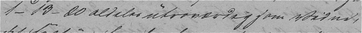1-13-20 aldeles utroværdig som Vidne,
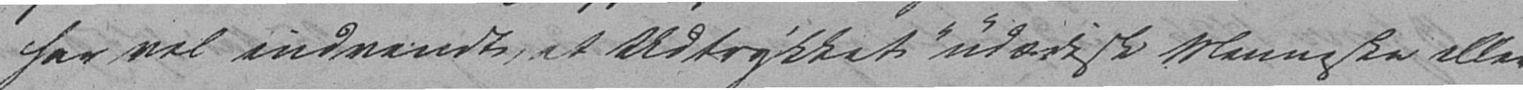har vel indvendt, at Udtrykket "udædisk Menneske eller
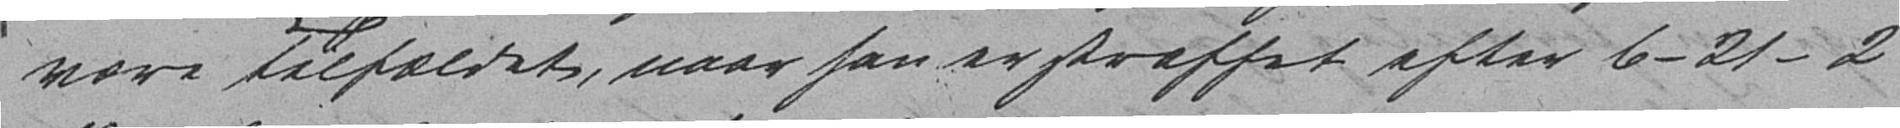være Tilfældet, naar han er straffet efter 6-21-2.
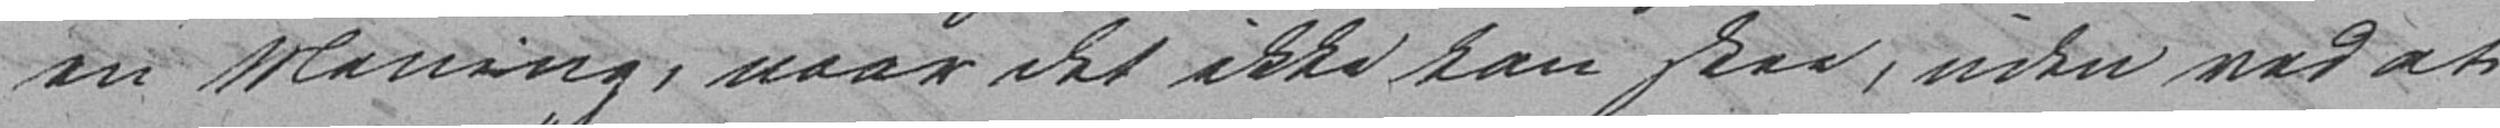en Mening, naar det ikke kan skee, uden ved at
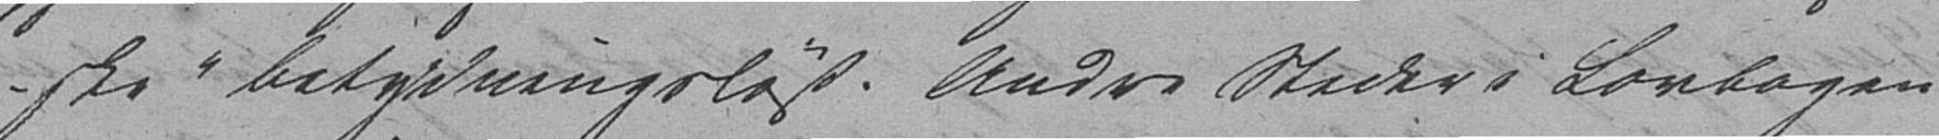ske" betydningsløs. Andre Steder i Lovbogen
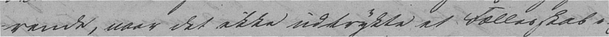vende, naar det ikke udtrykte et Fællesskab i
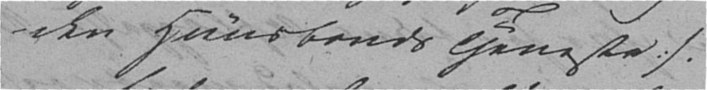den Husbonds Tjeneste :/.
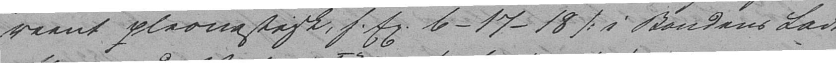rent pleonastisk, f. Ex. 6-17-18 /: i Bondens Lov
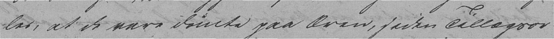les, at de vare dømte paa Æren, siden Tillægsor-
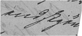endskjønt
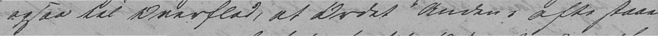ogsaa til Overflod, at Ordet "Anden" ofte staae
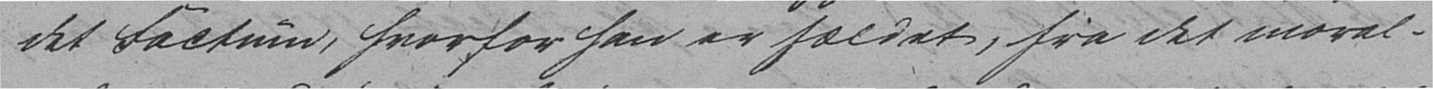det Factum, hvorfor han er fældet, fra det moral-
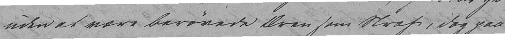uden at være berøvede Æren som Straf, dog paa
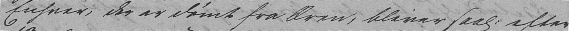Enhver, der er dømt fra Æren, bliver saal. efter
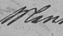Menne-
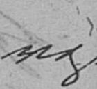viser
